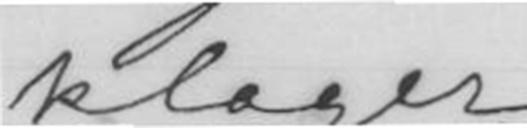klager,
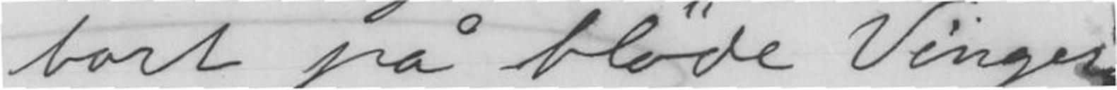bort på bløde Vinger!
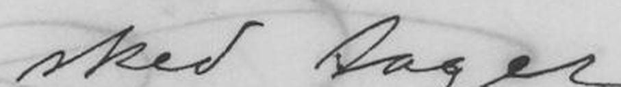sked tager
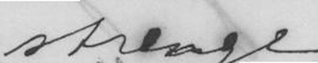strenge.
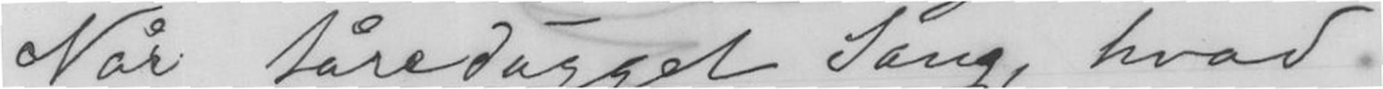Når tåredugget Sang, hvad
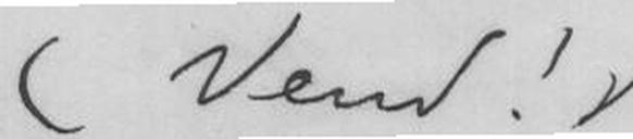(Vend!)
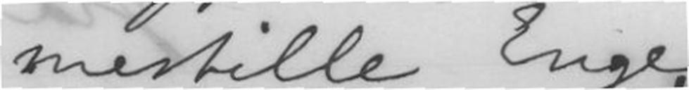mestille Enge.
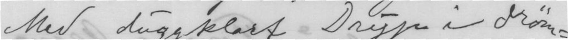Med duggklart Dryp i Drøm-
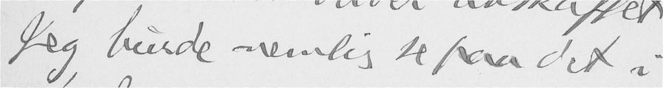Jeg burde nemlig se paa det i
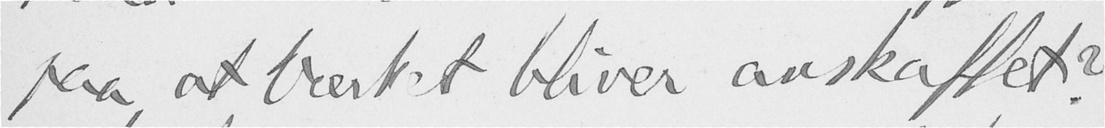paa, at værket bliver anskaffet?
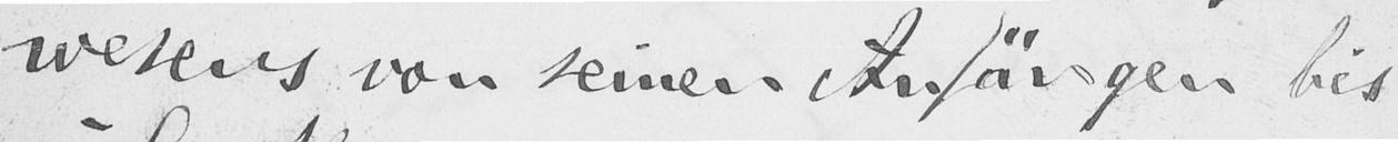wesens von seinen Anfängen bis
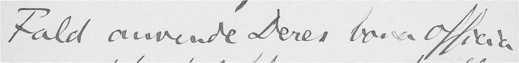Fald anvende Deres bona officia
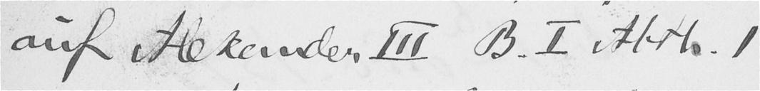auf Alexander III B. I Abth. 1
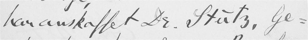har anskaffet Dr. Stutz, Ge-
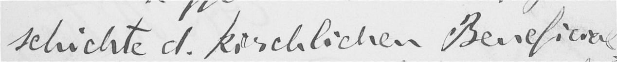schichte d. kirchlichen Beneficial-
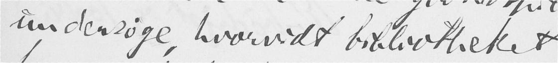undersøge, hvorvidt bibliotheket
